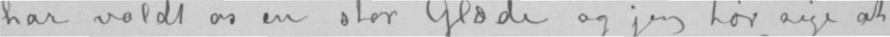har voldt os en stor Glæde og jeg tør sige at
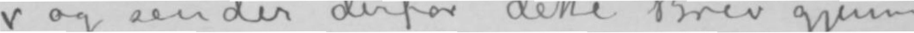og sender derfor dette Brev gjennem
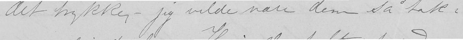det trykke, - jeg vilde være dem så tak-
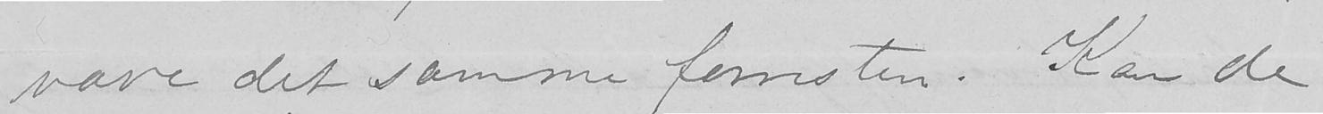være det samme forresten. Kan de
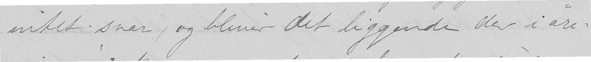intet svar, og bliver det liggende der i åre-
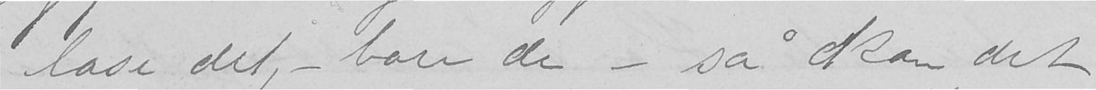læse det, - bare de - så kan det
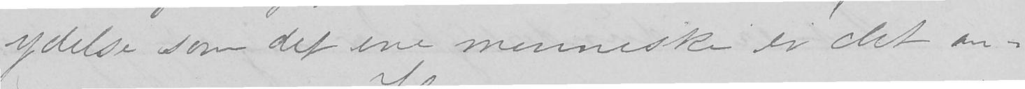ydelse som det ene menneske er det an-
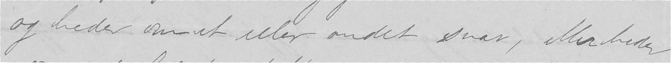og beder om et eller andet svar, eller beder
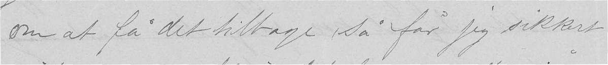om at få det tilbage så får jeg sikkert
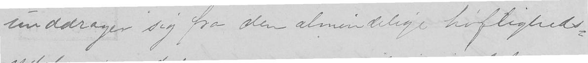unddrager sig fra den almindelige høfligheds-
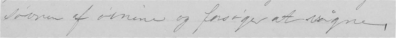søvnen af øinene og forsøger at vågne,
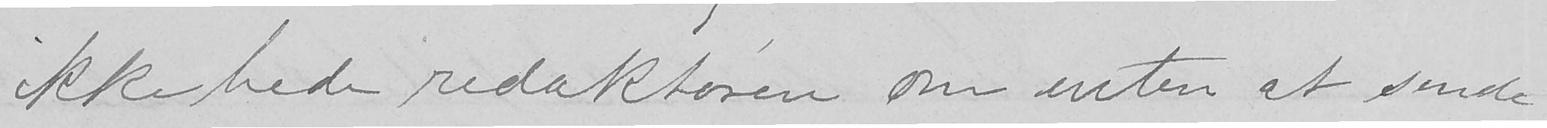ikke bede redaktøren om enten at sende
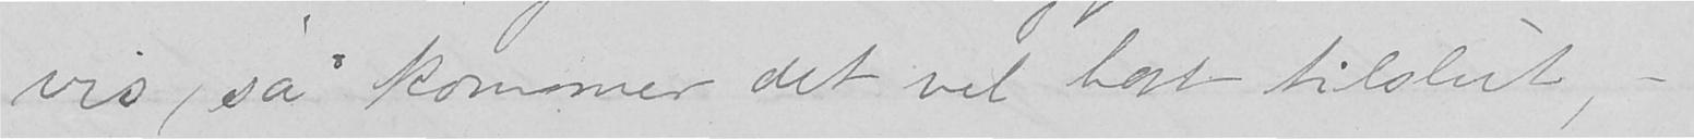vis, så kommer det vel bort tilslut, -
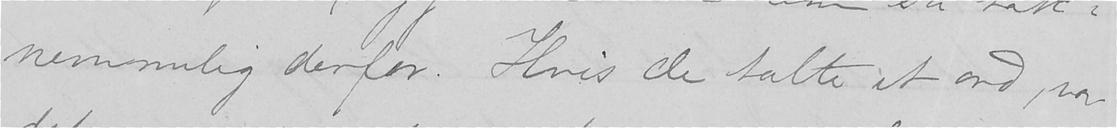nemmelig derfor. Hvis de talte et ord, var
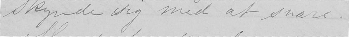skynde sig med at svare.
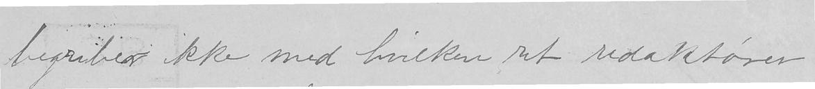begriber ikke med hvilken ret redaktører
